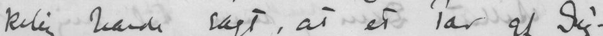kelig havde sagt, at et Par af Dig-
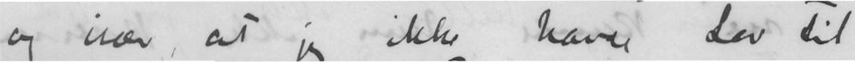og især at jeg ikke havde Lov til
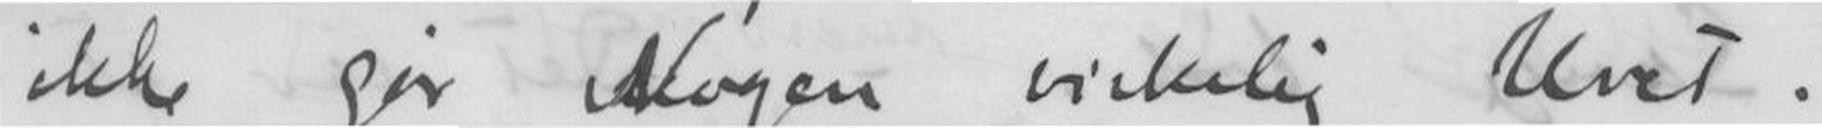ikke gir Nogen virkelig Uret.
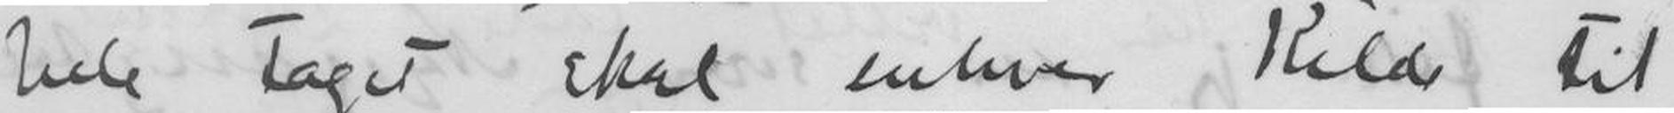hele taget skal enhver Kilde til
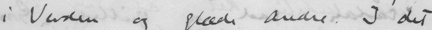i Verden og glæde andre. I det
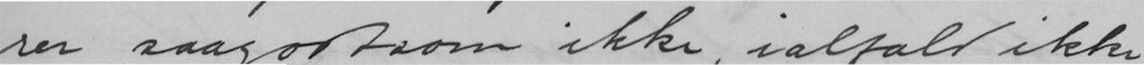rer saagodtsom ikke, ialfald ikke
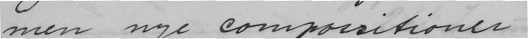men nye compoisitioner
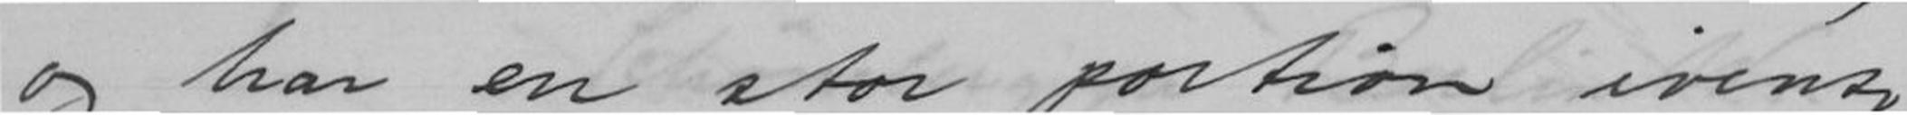og har en stor portion ivente,
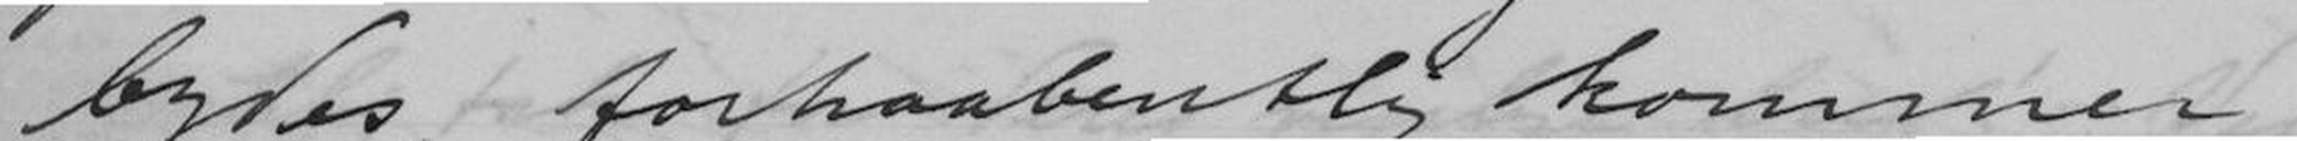bydes, forhaabentlig kommer
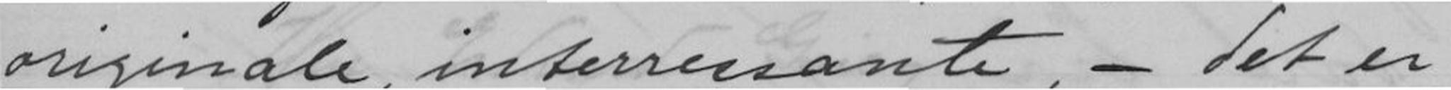originale, interressante, - det er
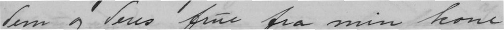til dem og deres frue fra min kone
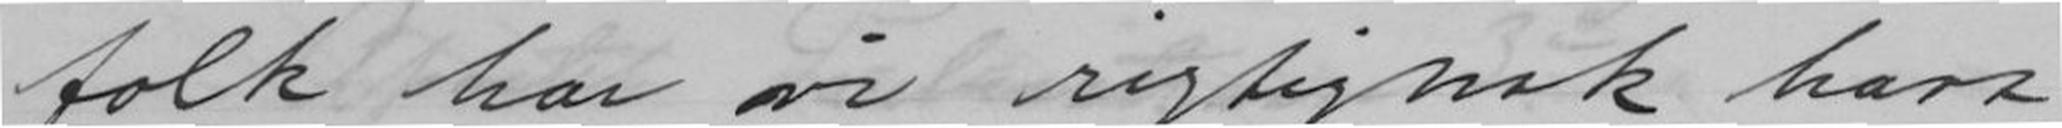folk har vi rigtignok havt,
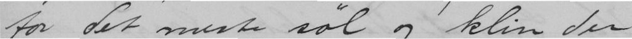for det meste søl og klin der
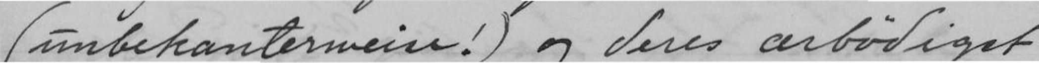(unbekanterweise!) og deres ærbødigst
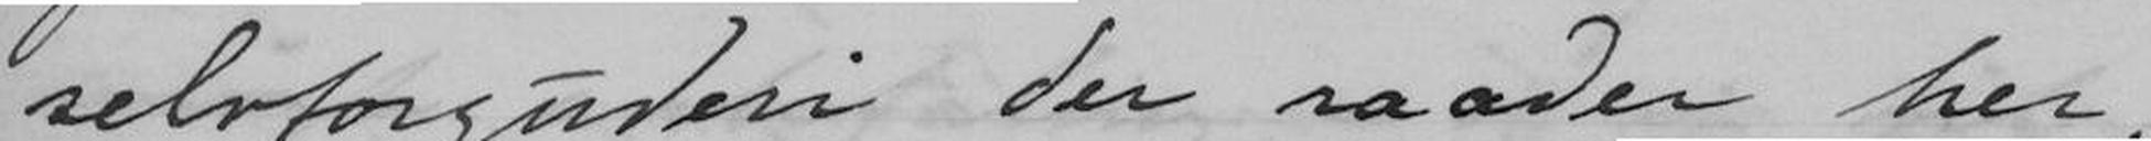selvforguderi der raader her,
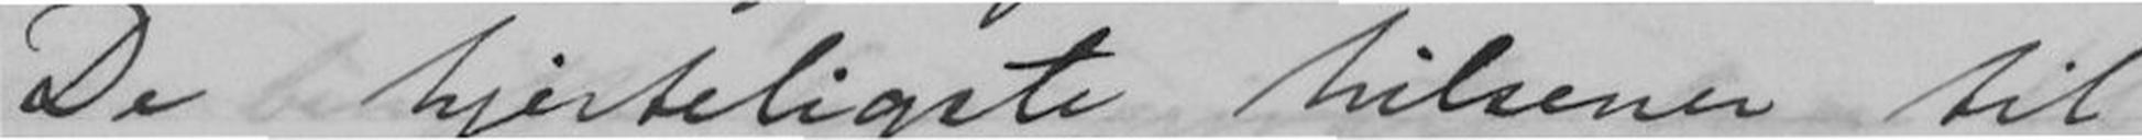De hjerteligste hilsener
